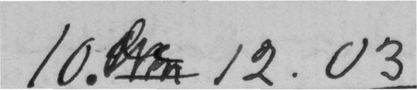10. 12.03
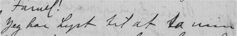Jeg har Lyst til at ta min
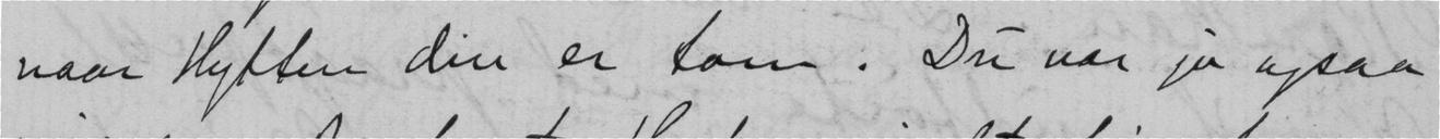naar Hytten din er tom. Du var jo ogsaa
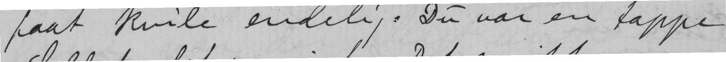faat kvile endelig. Du var en tapper
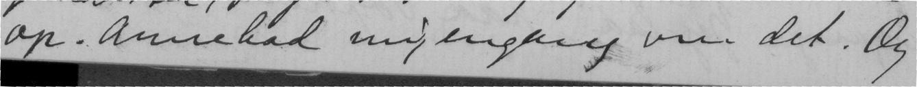op. Anne bad mig engang om det. Og
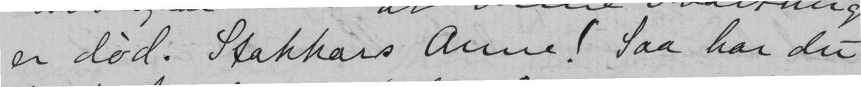er død. Stakkars Anne! Saa har du
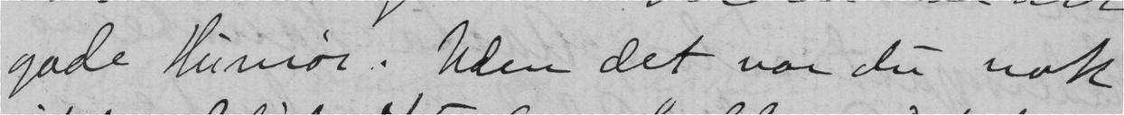gode Humör. Uden det var du nok
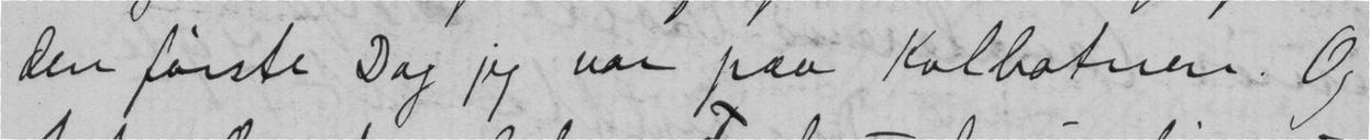den förste Dag jeg var paa Kolbotnen. Og
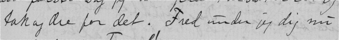tak og ære for det. Fred under jeg dig nu
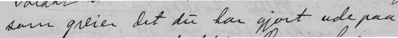som greier det du har gjort ude paa
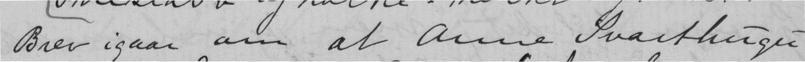Brev igaar om at Anne Svarthugu
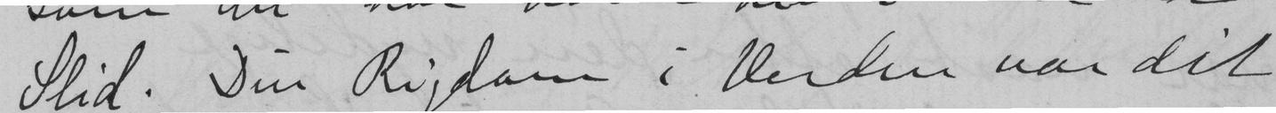Slid. Din Rigdom i Verden var dit
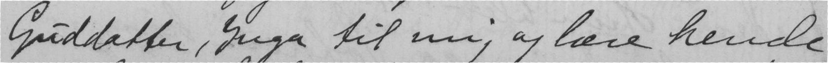Guddatter, Inga til mig og lære hende
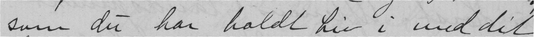som du har holdt Liv i med dit
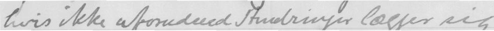hvis ikke uforudseede Hindringer lægger sig
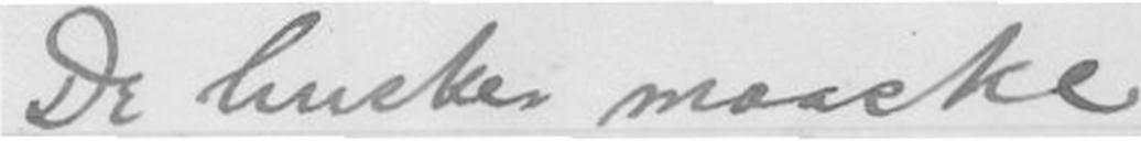De husker maaske,
